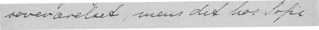soveværelset, mens det hos Sofie
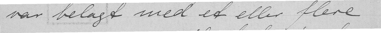var belagt med et eller flere
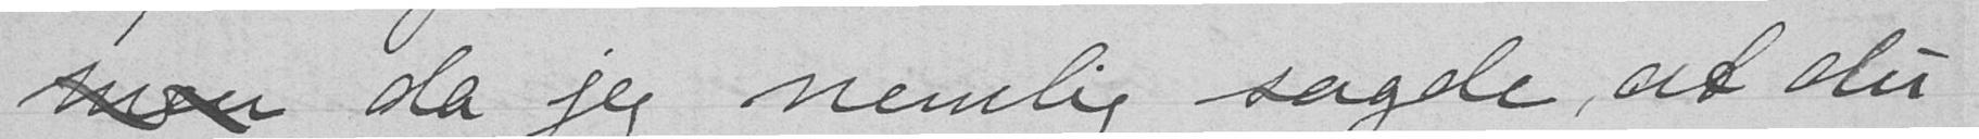men da jeg nemlig sagde, at du
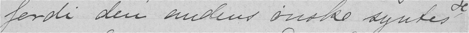fordi den andens ønske syntes
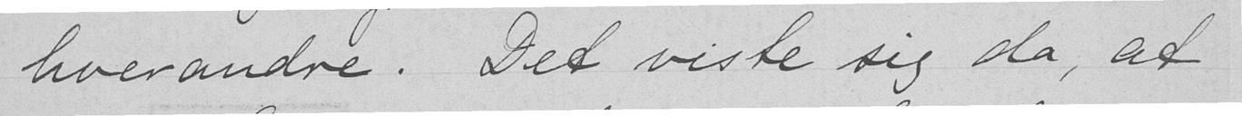hverandre. Det viste sig da, at
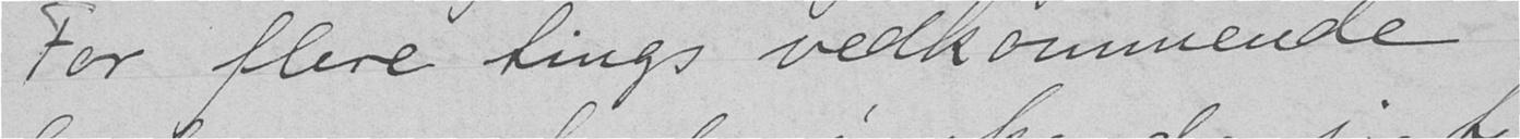For flere tings vedkommende
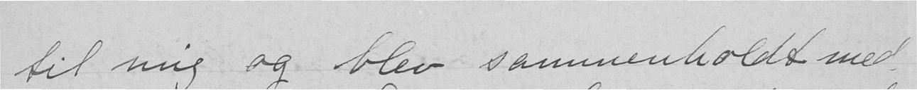til mig og blev sammenholdt med
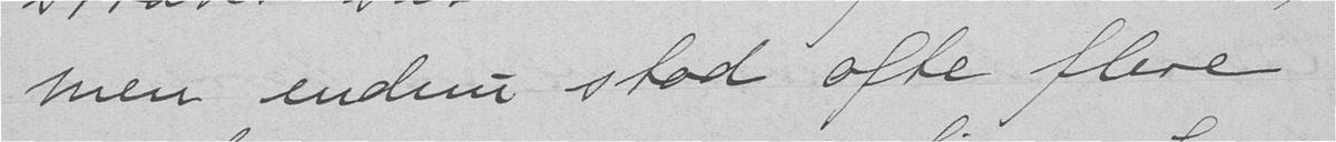men endnu stod ofte flere
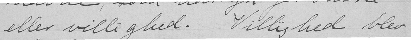eller villighed. Villighed blev
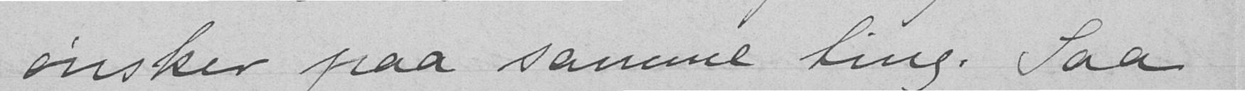ønsker paa samme ting. Saa
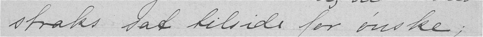straks sat tilside for ønske;
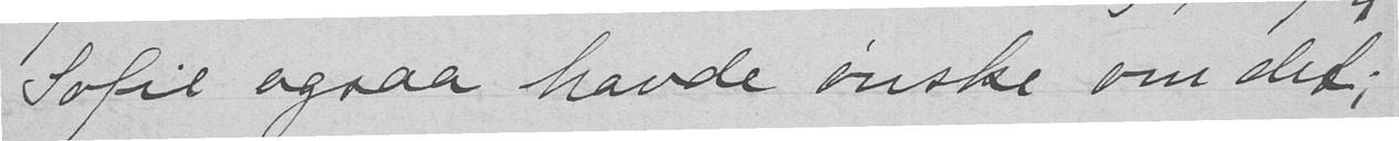Sofie ogsaa havde ønske om det;
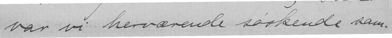var vi herværende søskende sam-
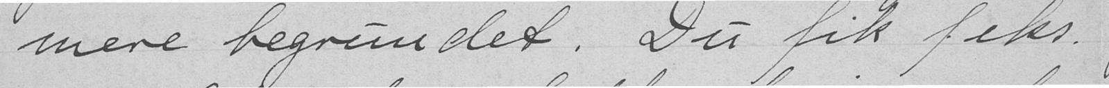mere begrundet. Du fik feks.
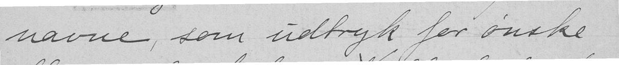navne, som udtryk for ønske
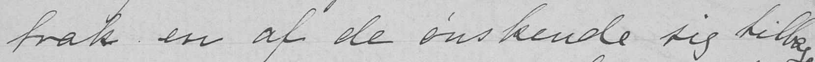trak en af de ønskende sig tilbage,
